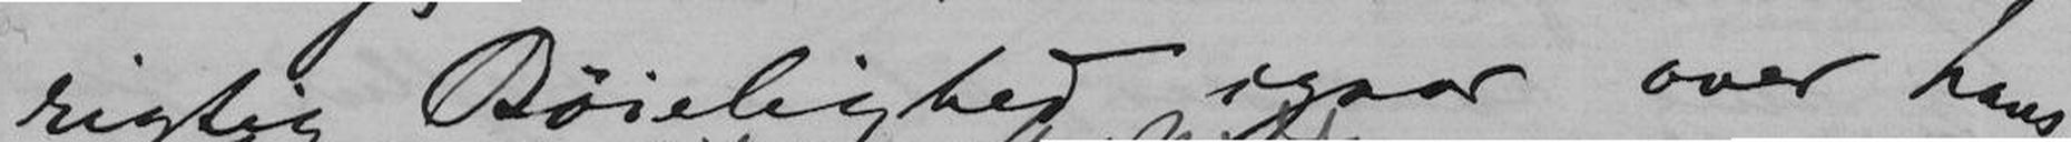rigtig Bøielighed igaar over hans
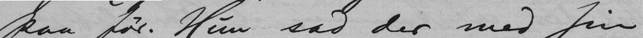paa før. Hun sad der med sin
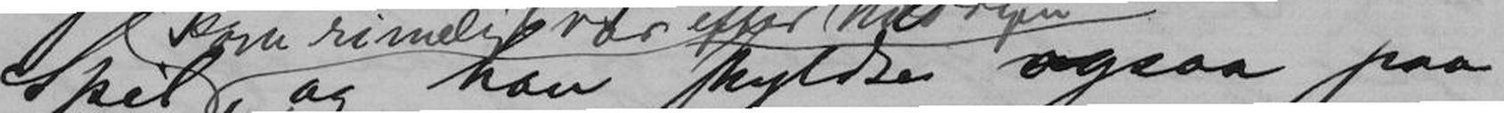Spil , og han skyldte ogsaa paa
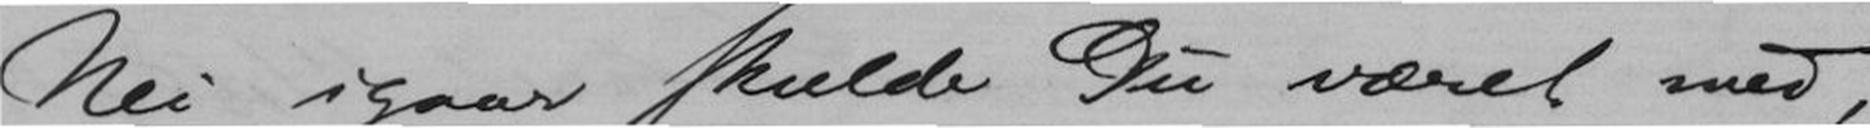Nei igaar skulde Du været med,
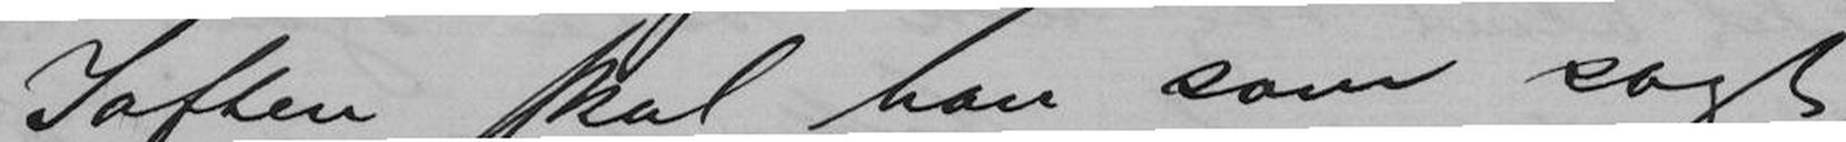Iaften skal han som sagt
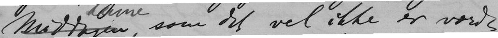Middagen, som det vel ikke er værdt
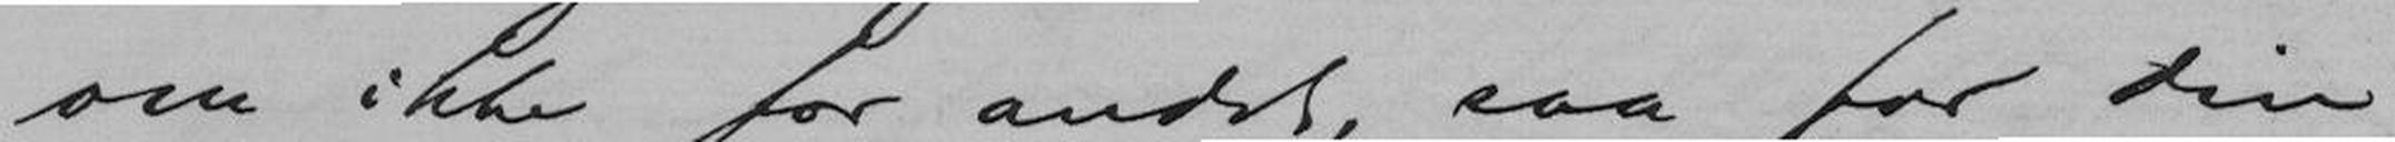om ikke for andet, saa for din
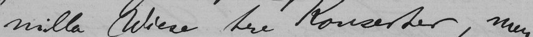illa Wiese tre Konserter, men
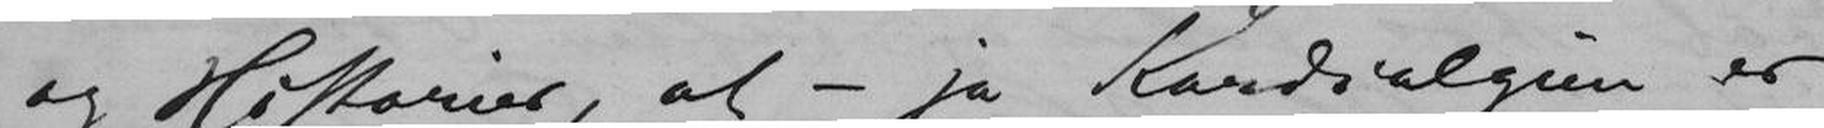og Historier, at - ja Kardialgien er
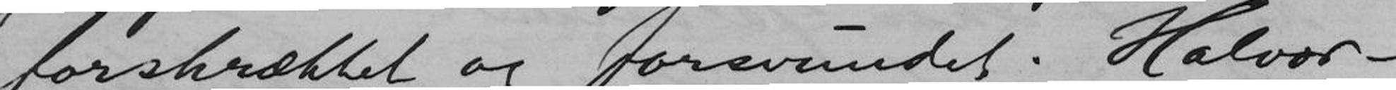forskrækket og forsvundet. Halvor-
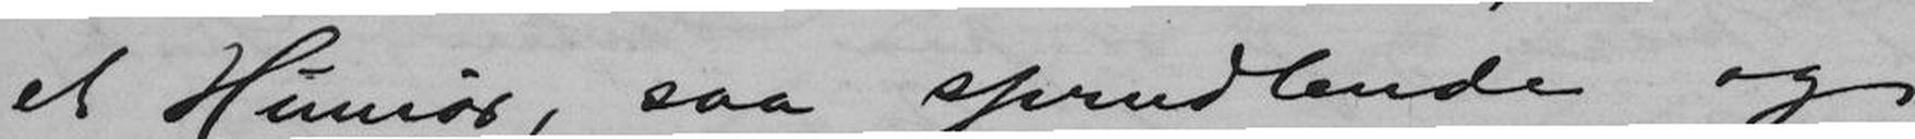et Humør, saa sprudlende og
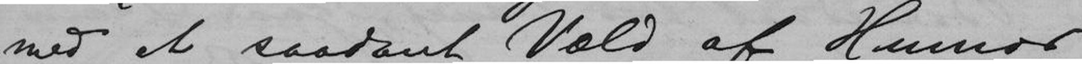med et saadant Væld af Humor
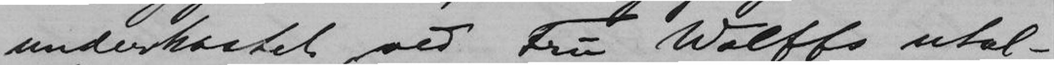underkastet ved Fru Wolffs utal-
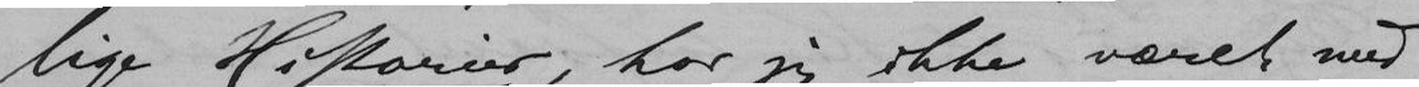lige Historier, har jeg ikke været med
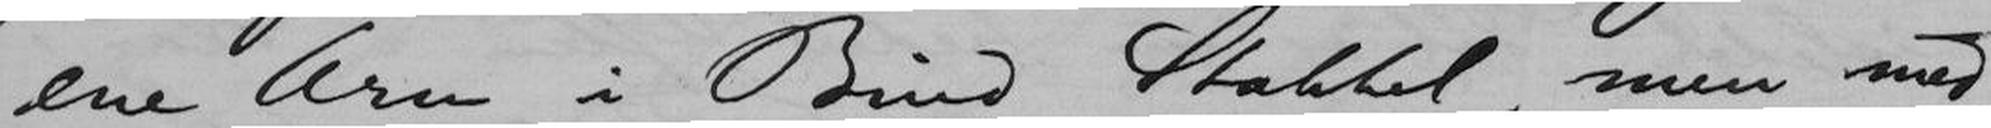ene Arm i Bind Stakkel, men med
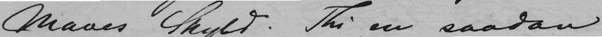Maves Skyld. Thi en saadan
